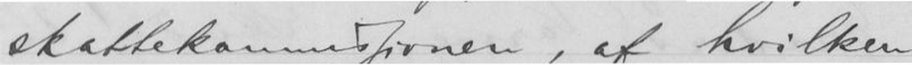skattekommissionen, af hvilken
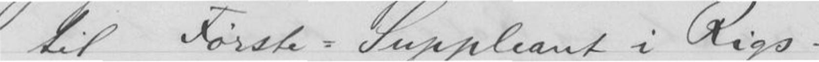til Første=Suppleant i Rigs-
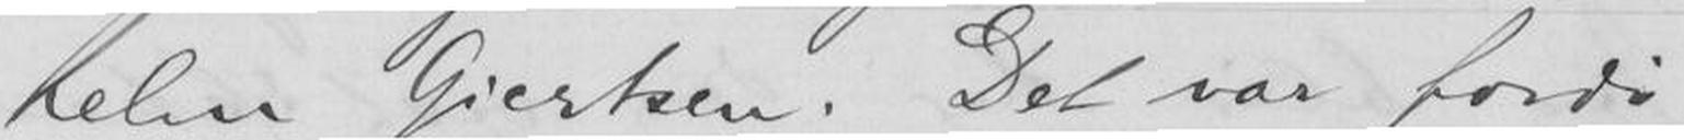helm Giertsen. Det var fordi
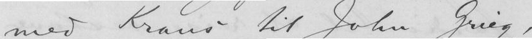med Krans til John Grieg,
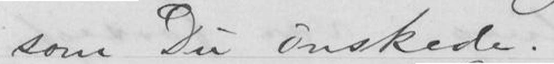som Du ønskede. -
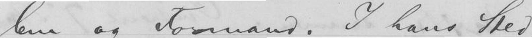lem og Formand. I hans Sted
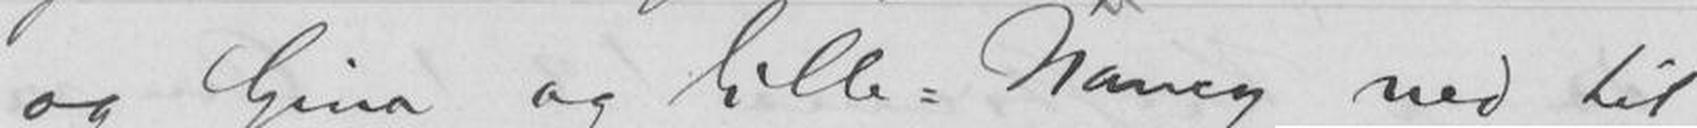og Gina og lille=Nancy ned til
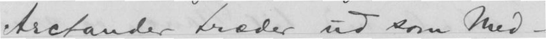Arctander træder ud som Med-
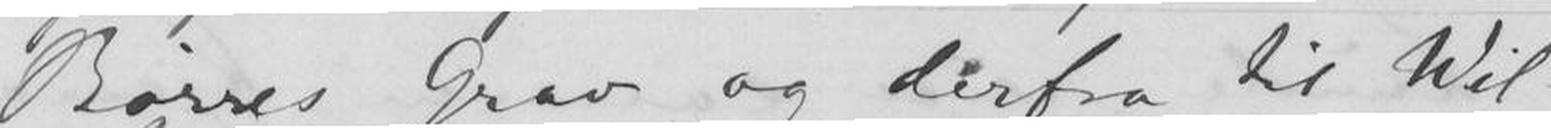Børres Grav og derfra til Wil-
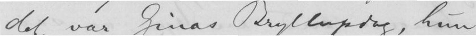det var Ginas Bryllupsdag, hun
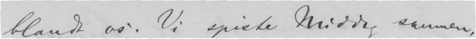blandt os. Vi spiste Middag sammen,
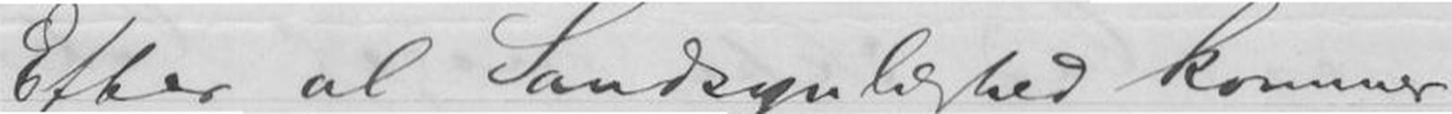Efter al Sandsynlighed kommer
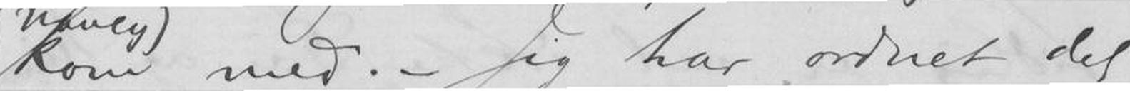kom med. - Jeg har ordnet det
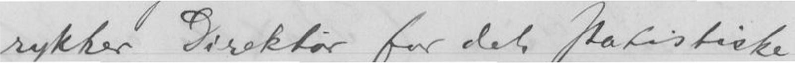rykker Direktør for det statistiske
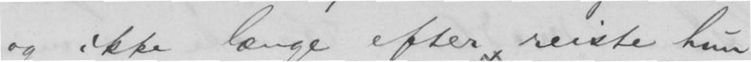og ikke længe efter reiste hun
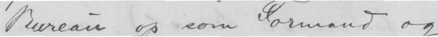Bureau op som Formand og
